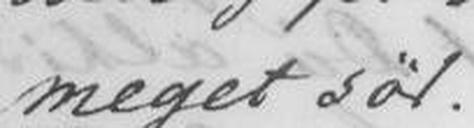meget sød.
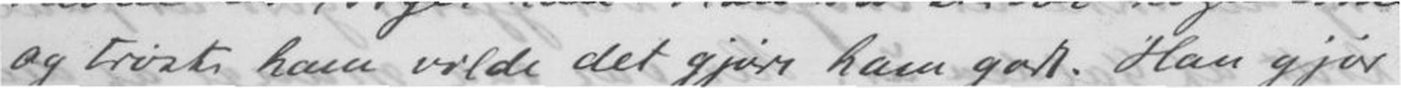og trøste ham vilde det gjøre ham godt. Han gjør
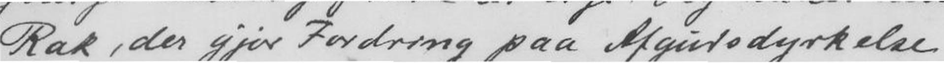Rak, der gjør Fordring paa Afgudsdyrkelse
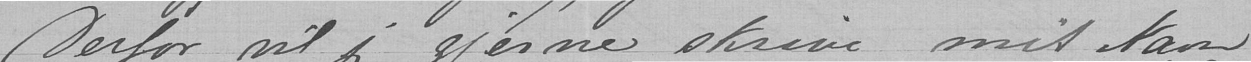Derfor vil jeg gjerne skrive mit Navn
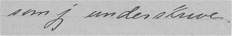som jeg underskriver.
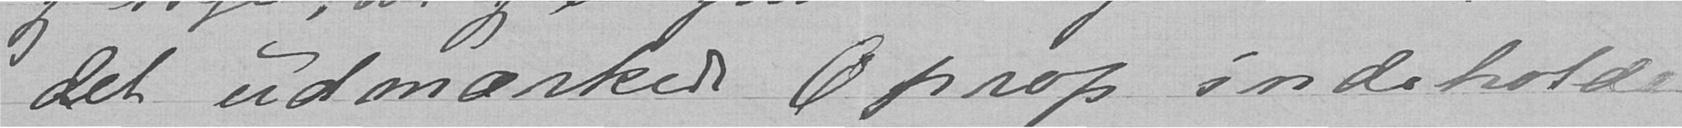det udmærkede Oprop indeholde
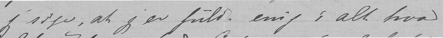jeg sige, at jeg er fuldt enig i alt hvad
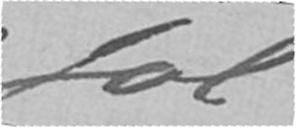fol
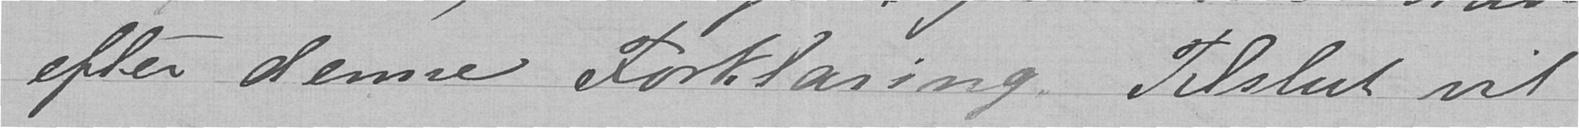efter denne Forklaring. Tilslut vil
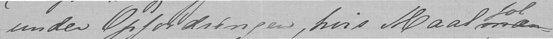under Opfordringen, hvis Maalmæn
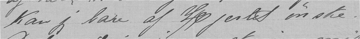kan jeg bare af Hjertet ønske.
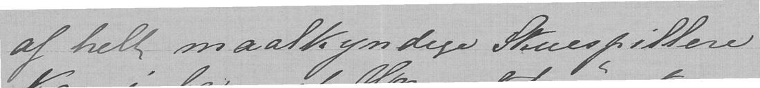af helt maalkyndige Skuespillere
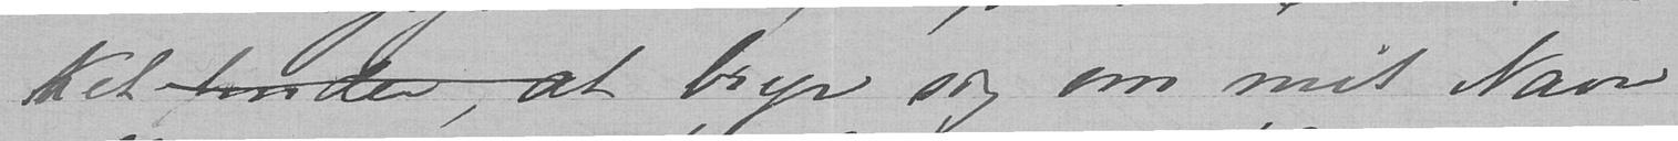ket finder at bryr sig om mit Navn
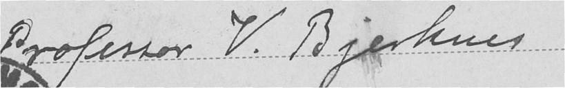Professor V. Bjerknes
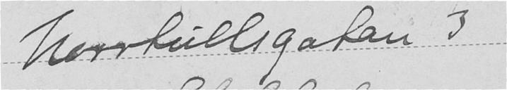Norrtullsgatan 3
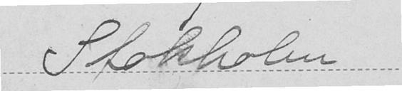Stokholm
